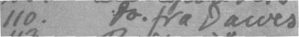110. do. fra i Dawes
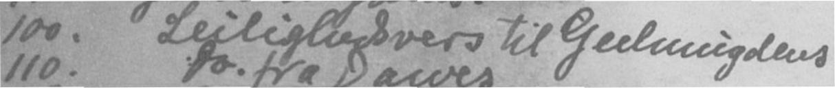100. Leilighedsvers til Geelmuydens
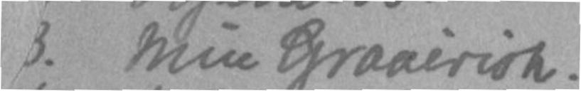3. Min Graairisk.
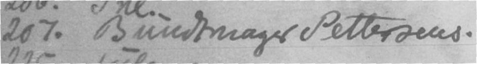207. Bundtmager Pettersens.
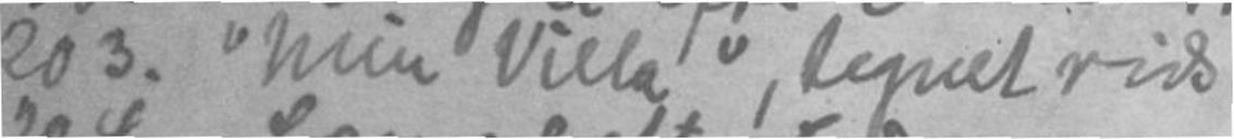203. "Min Villa", tegnet rids
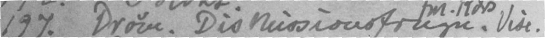197. Drøm. Diskussionsfrngn. frk. Rds. Vise.
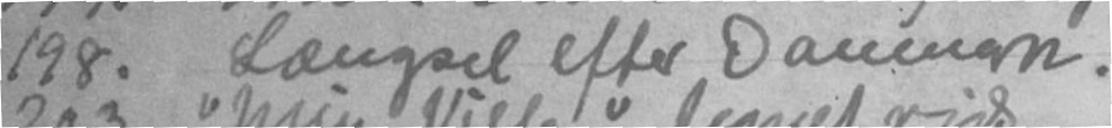198. Længsel efter Danmark.
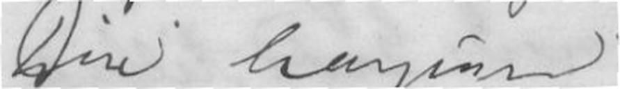Din hengivne
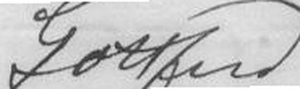Gottfred
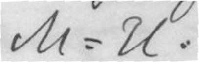M-H.
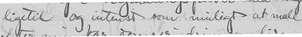ligetil og intenst som muligt at male
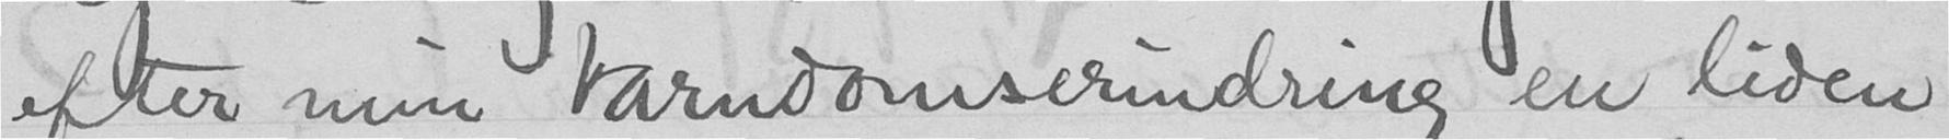efter min barndomserindring en liden
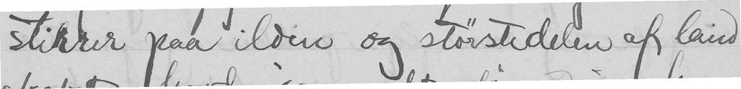stirrer paa ilden og størstedelen af land-
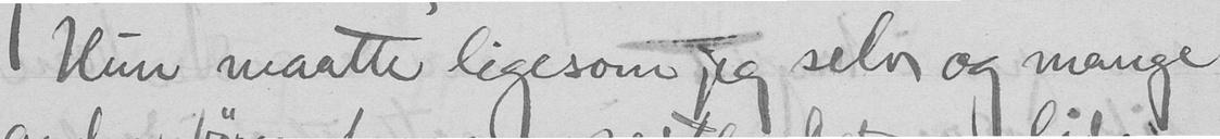Hun - maatte ligesom jeg selv og mange
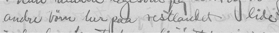andre børn her paa vestlandet lide
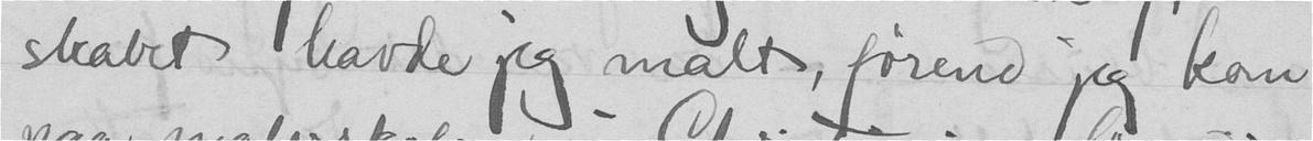skabet havde jeg malt, førend jeg kom
